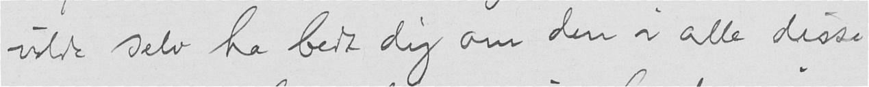vilde selv ha bedt dig om den i alle disse
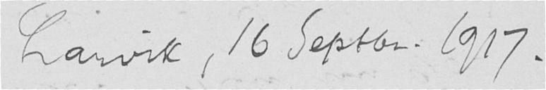Larvik, 16 Septbr. 1917.
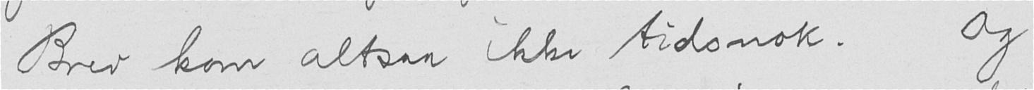Brev kom altsaa ikke tidsnok. Og
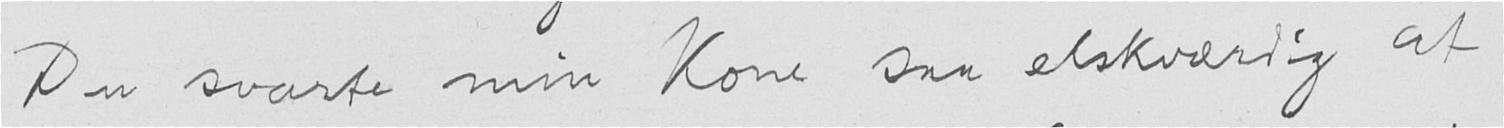Du svarte min Kone saa elskværdig at
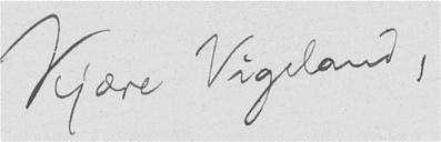Kjære Vigeland,
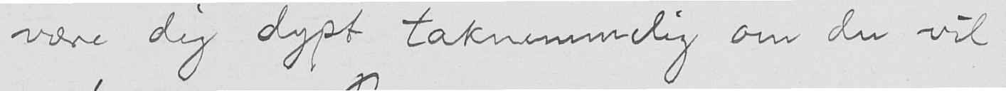være dig dypt taknemmelig om du vil
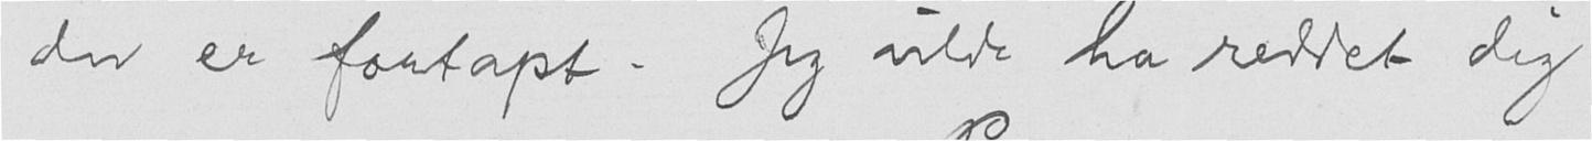du er fortapt. Jeg vilde ha reddet dig
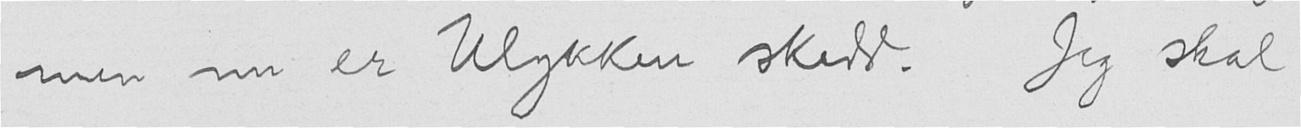men nu er Ulykken skedd. Jeg skal
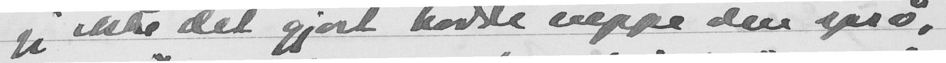jeg ikke det gjort hadde neppe den sprø,
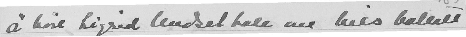Å høre Sigrid Undset tale om Nils Collett
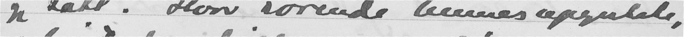jeg satt. Hvor rørende hennes oprystete,
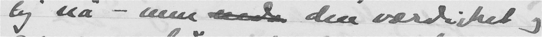lig nå - men  den værdighet og
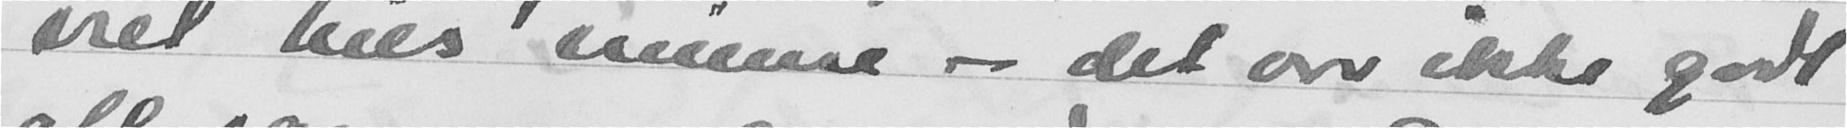viet Nils' minne - det var ikke godt
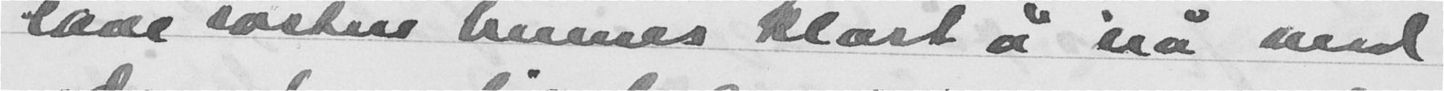lave søsteren hennes klart å nå med
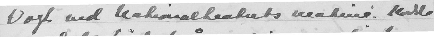Vogt ved Nationalteatrets matene. Hadde
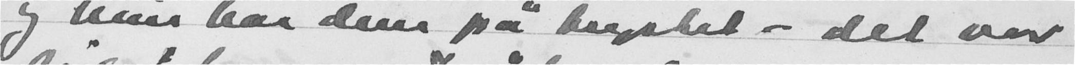og hun bar dem på brystet - det var
